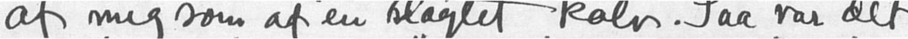af mig som af en slagtet kalv. Saa var det
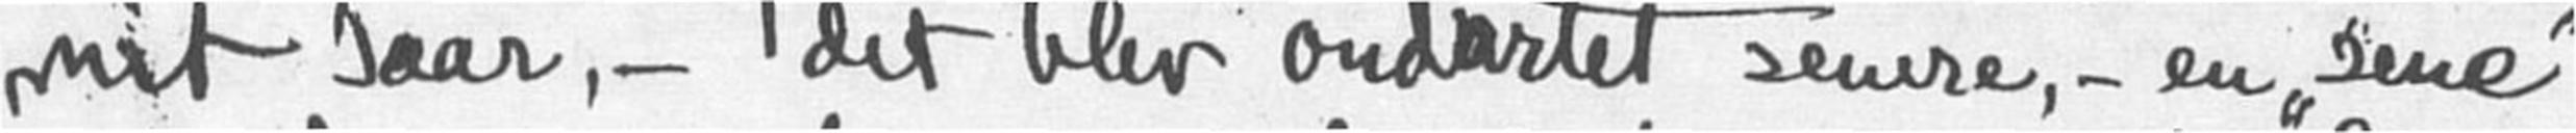mit saar, - det blev ondartet senere, - en "sene"
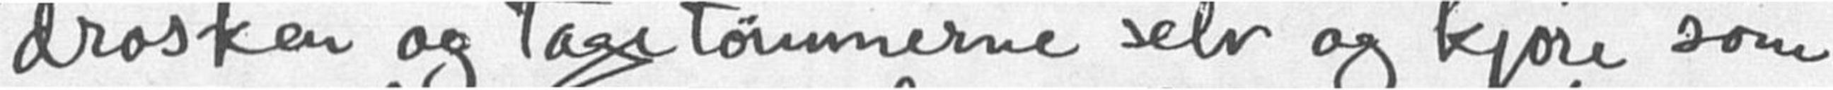drosken og tage tømmerne selv og kjøre som
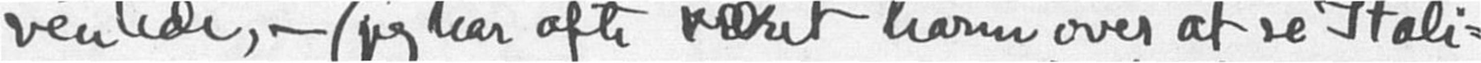ventede, - (jeg har ofte været harm overat se Itali-
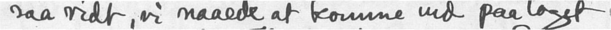saa vidt, vi naaede at komme ind paa toget i
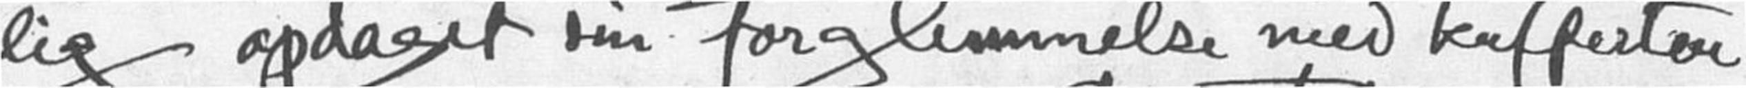lig opdaget sin forglemmelse med kufferten
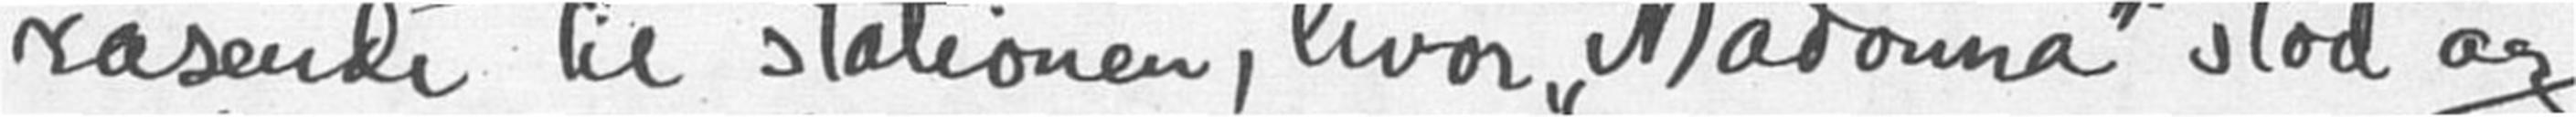rasende til stationen, hvor "Madonna" stod og
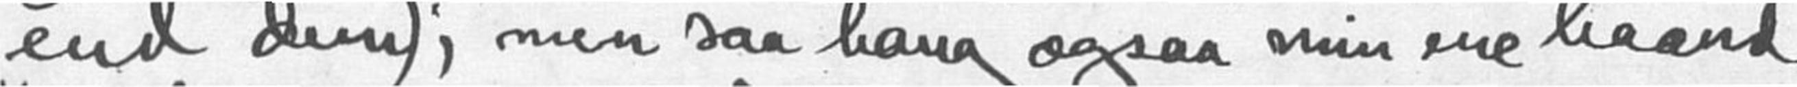end dem); men saa hang ogsaa min ene haand
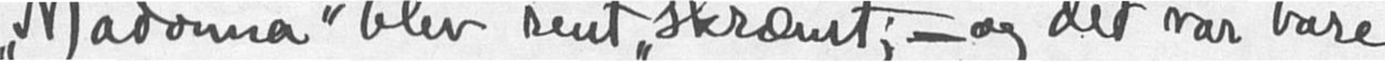"Madonna" blev rent "skræmt"; - og det var bare
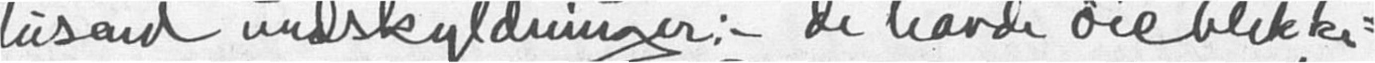tusend undskyldninger: - de havde øieblikke-
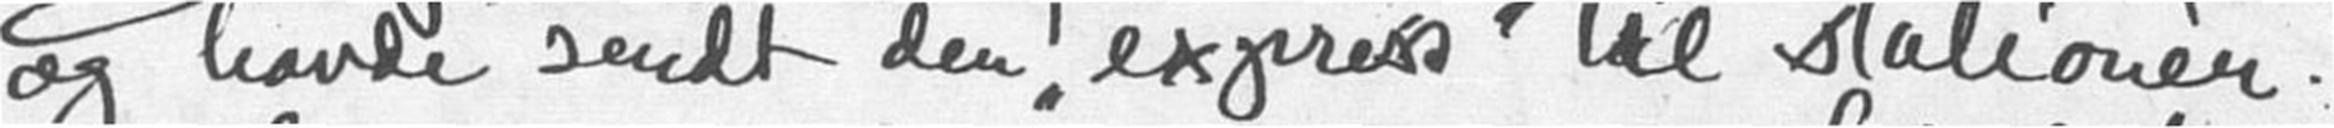og havde sendt den "express" til stationen.-
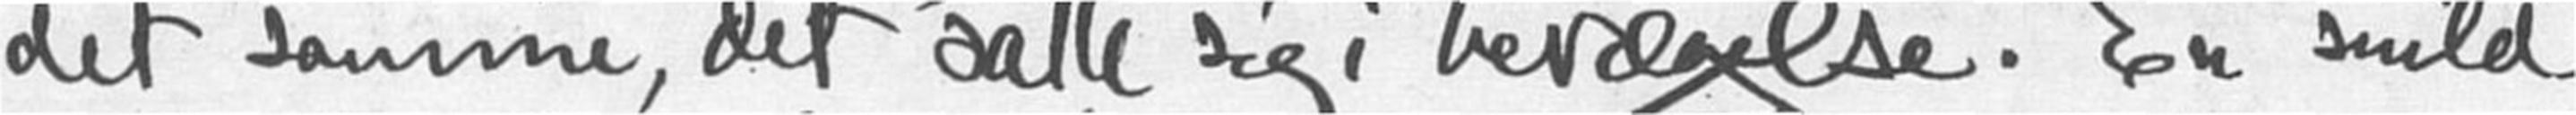det samme, det satte sig i bevægelse. En snild
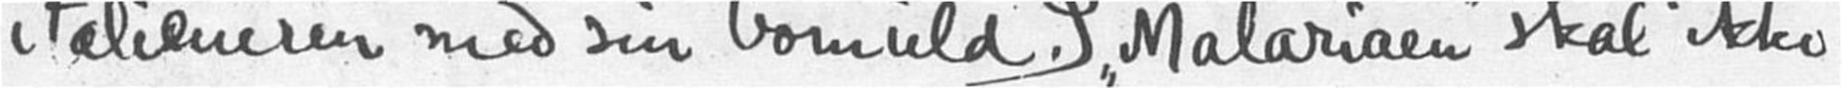italieneren med sin bomuld). "Malariaen" skal ikke
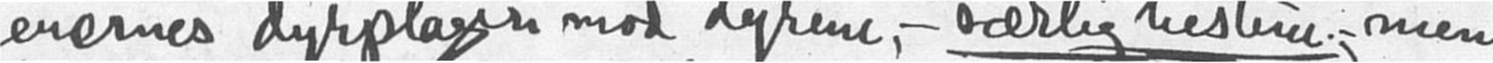enernes dyrplageri mod dyrene, - særlig hesten; - men
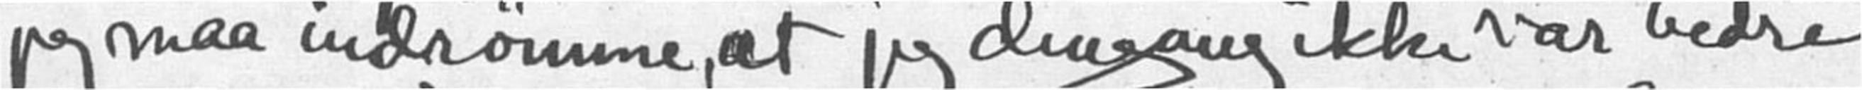jeg maa indrømme, at jeg dengang ikke var bedre
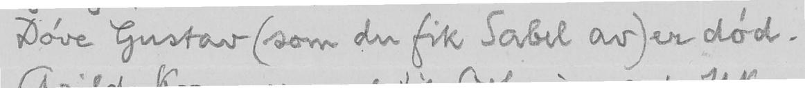Døve Gustav (som du fik Sabel av) er død.
